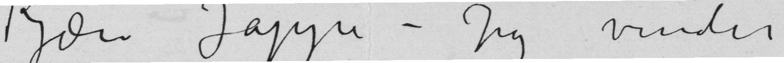Kjære Jappe – Jeg vinder
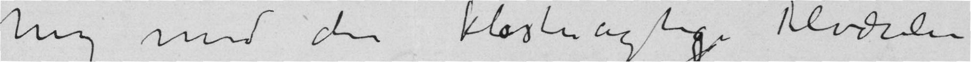mig med den klosteragtige Tilværelse
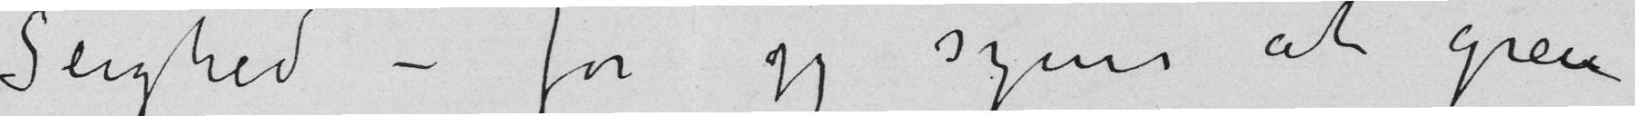Seighed – for jeg synes at greie
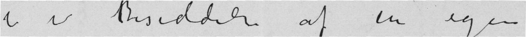er i Besiddelse af en egen
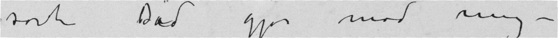sorte Død gjør mod mig –
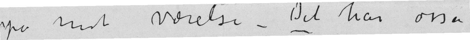på mit værelse – Det har osså
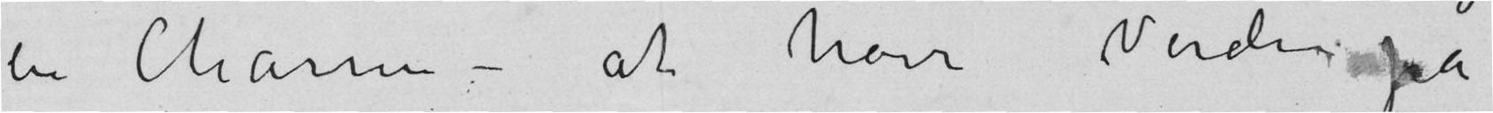sin Charme – at have Verden på
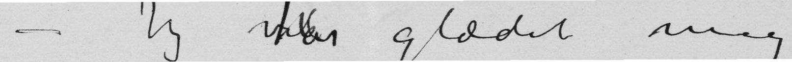– Jeg har glædet mig
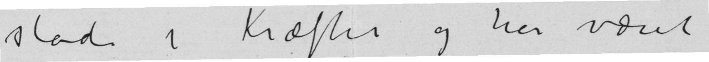stadi i Kræfter og har været
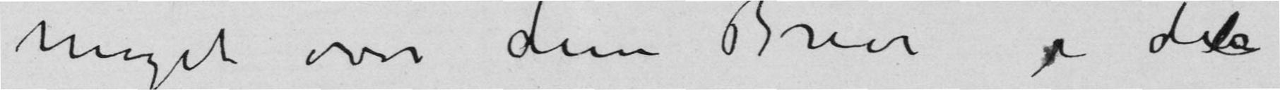meget over dine Breve de
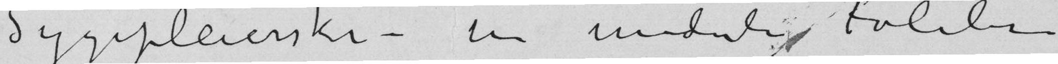Sygepleierske – en underlig Følelse
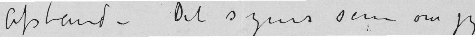Afstand – Det synes som om jeg
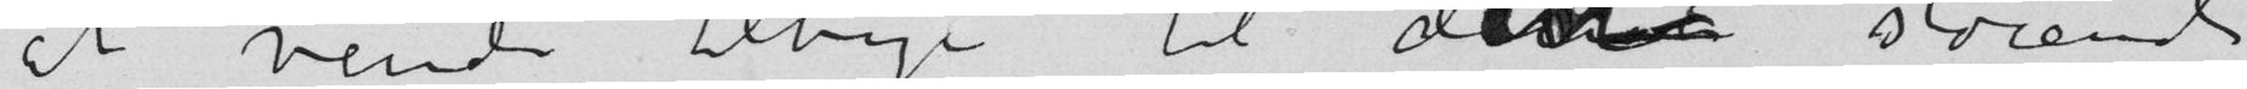at vende tilbage til den støiende
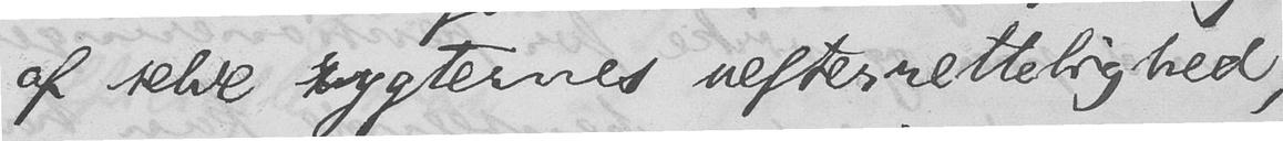af selve rygternes efterrettelighed,
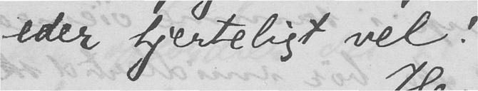eder hjerteligt vel!
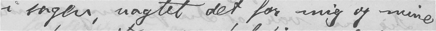i sagen, uagtet det for mig og mine
